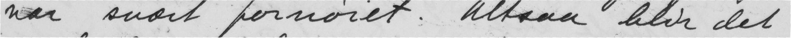var svært fornøiet. Altsaa blir det
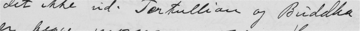det ikke ud. Tertullian og Buddha
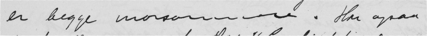er begge morsommere. Har ogsaa
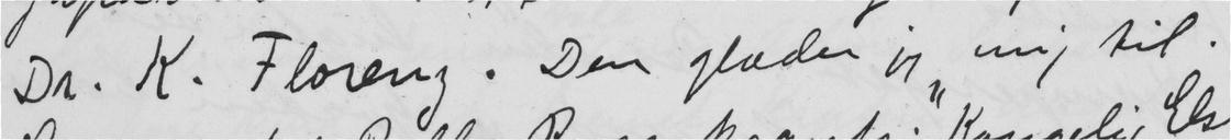Dr. K. Florenz. Den glæder jeg mig til.
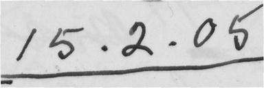15.2.05
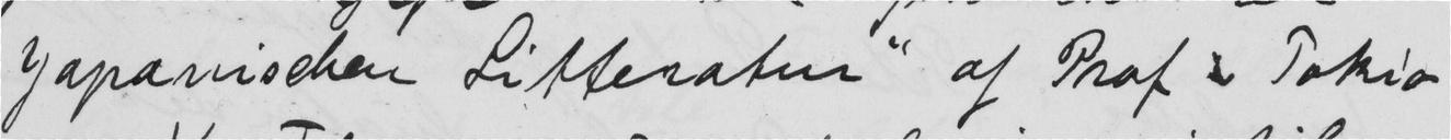Japanischen Litteratur" af Prof i Tokio
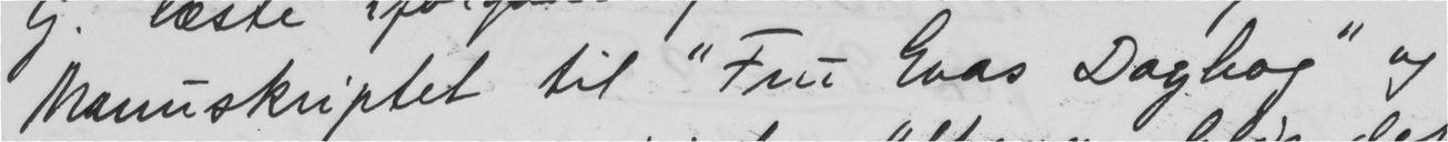Mannskriptet til "Fru Evas Dagbog" og
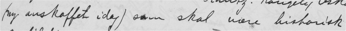(ny anskaffet idag) som skal være historisk
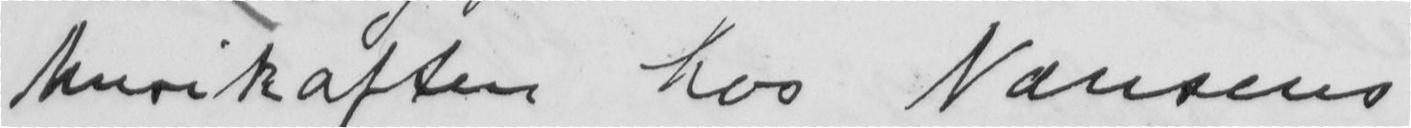Musikaften hos Nansens
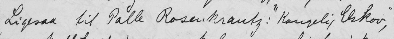Ligesaa til Palle Rosenkrantz: "Kongelig Eskov",
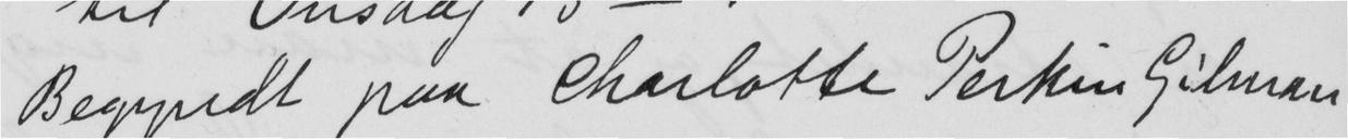Begyndt paa Charlotte Perkin Gilman
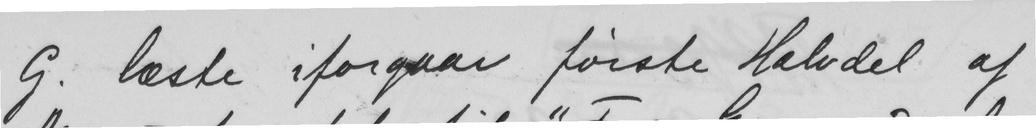G. læste iforgaar første Halvdel af
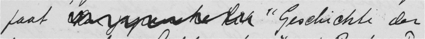faat  "Geschichti der
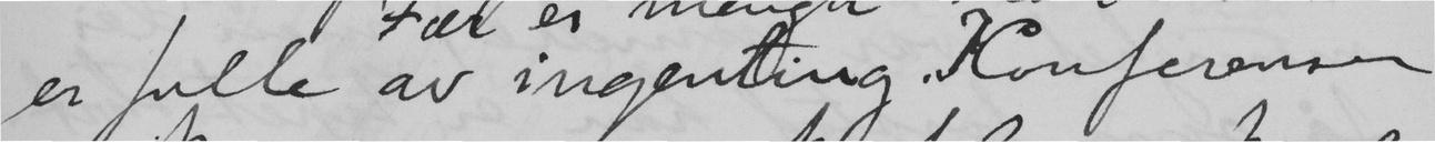er fulle av ingenting. Konferenser
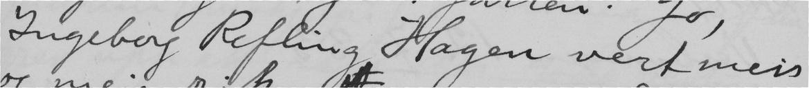Ingeborg Refling Hagen vert meir
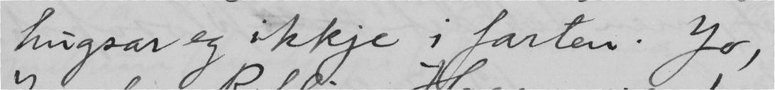hugsar eg ikkje i farten. Jo,
